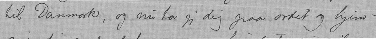til Danmark, og nu tar jeg dig paa ordet og hjem-
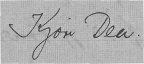Kjære Dea!
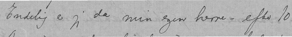Endelig er jeg da min egen herre — efter 10
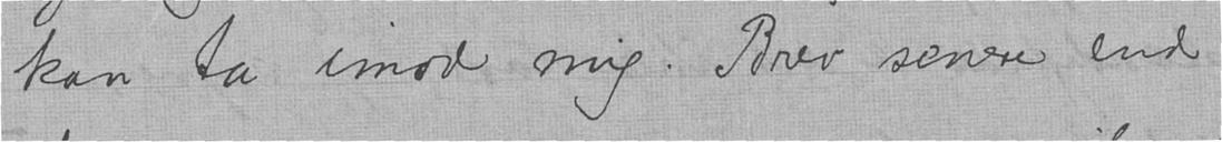kan ta imod mig. Brev senere end
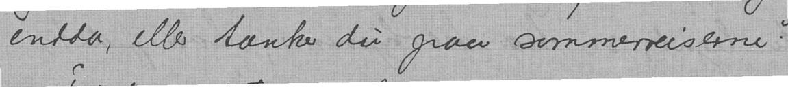endda, eller tænker du paa sommerreiserne?
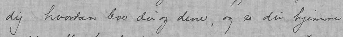dig — hvordan lever du og dine, og er du hjemme
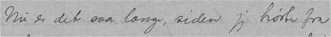Nu er det saa længe, siden jeg hørte fra
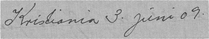Kristiania 3. juni 09.
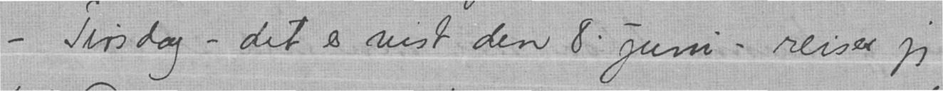— Tirsdag — det er vist den 8. juni — reiser jeg
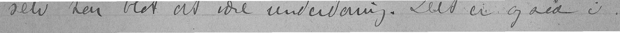selv har blot at være underdanig. Det er ogsaa i
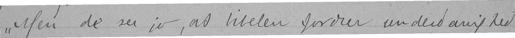"Men de ser jo, at bibelen fordrer underdanighed
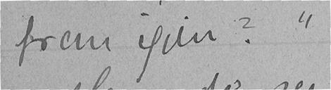frem igjen?"
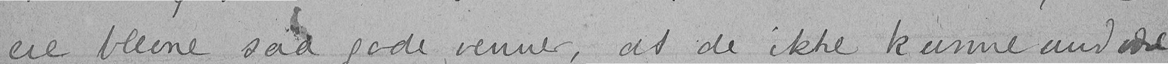ere blevne saa gode venner, at de ikke kunne undvære
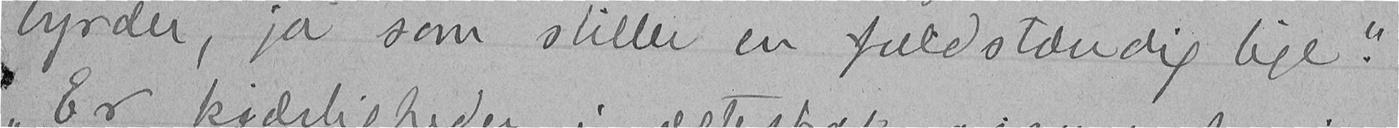byrder, ja som stiller en fuldstændig lige."
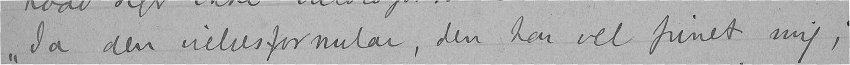"Ja den vielesformular, den har vel pinet mig,
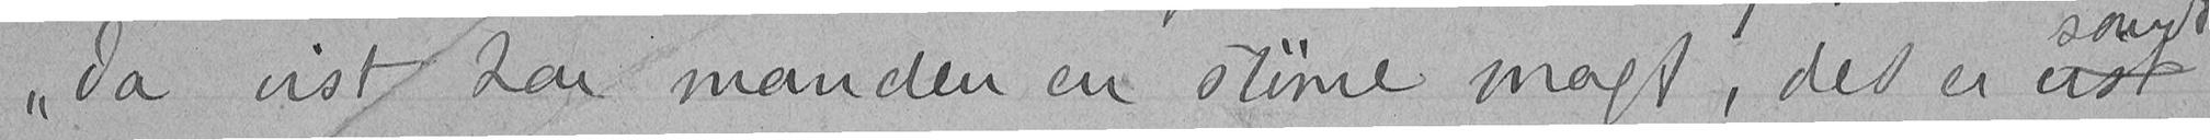"Ja vist har manden en større magt, det er vist
